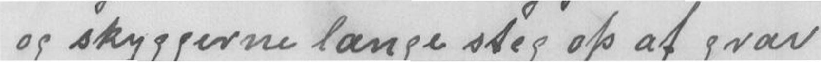og skyggerne lange steg op af grav
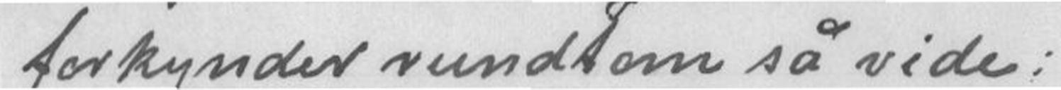forkynder rundtom så vide:
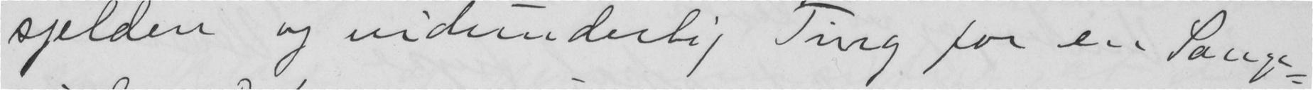sjelden og vidunderlig Ting for en Sange-
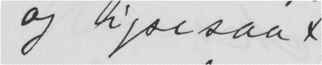og ligesaa
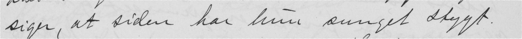siger, at siden har hun sunget stygt.
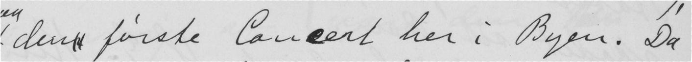den første Concert her i Byen. Da
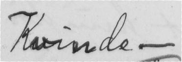Kvinde -
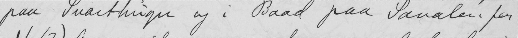paa Svarthuger og i Baad paa Savalen for
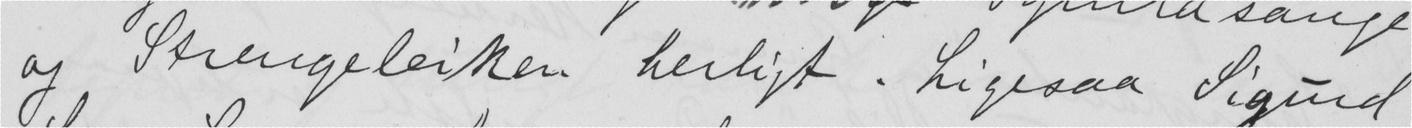og Strengeleiken herligt. Ligesaa Sigurd
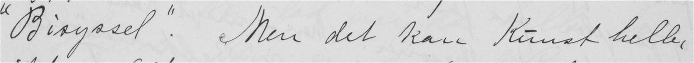"Bisyssel". Men det kan Kunst heller
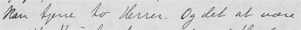kan tjene to Herrer. Og det at være
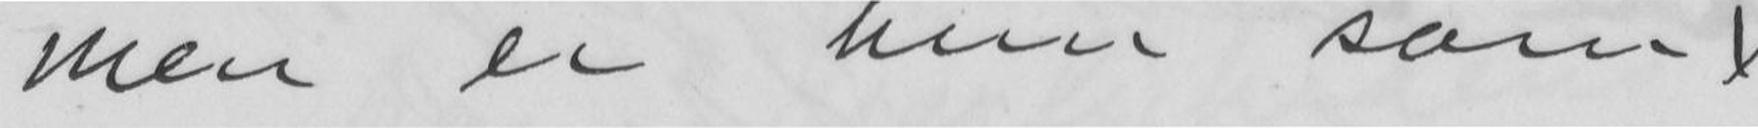Men er hun som D-, saa er hun
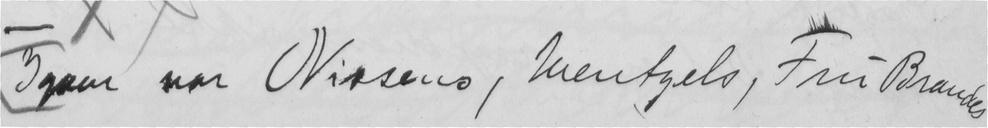Igaar var Nissens, Wentzels, Fru Brandes
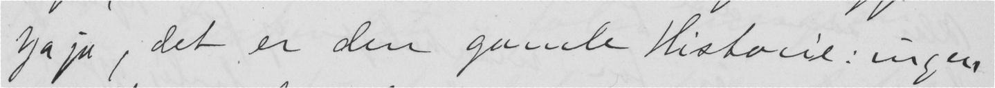Ja ja, det er den gamle Historie: ingen
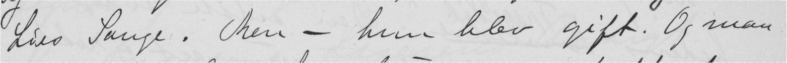Lies Sange. Men - hun blev gift. Og man
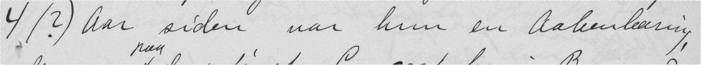4 (?) Aar siden var hun en Aabenbaring
